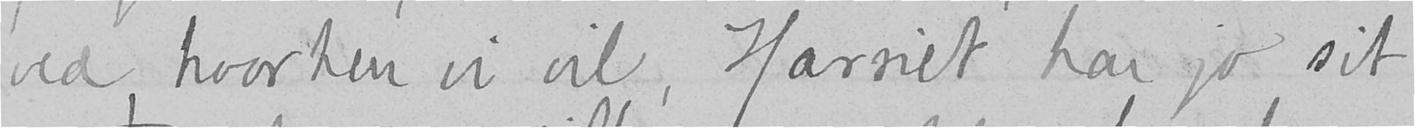ved, hvorhen vi vil, Harriet har jo sit
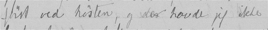først ved høsten, og der havde jeg ikke
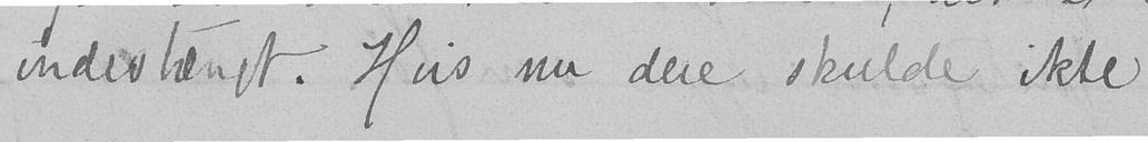indestængt. Hvis nu dere skulde ikke
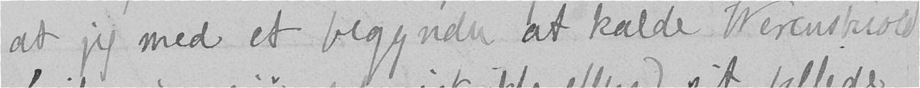at jeg med et begynder at kalde Werenskiold
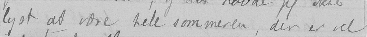lyst at være hele sommeren, der er vel
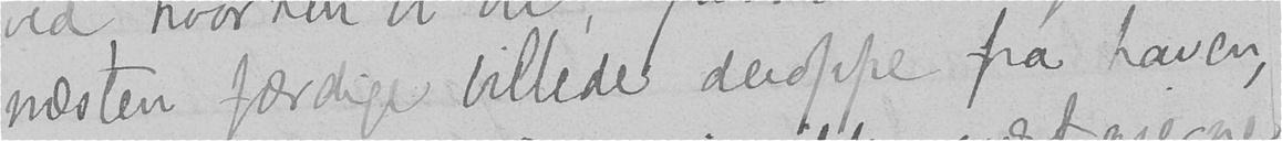næsten færdige billede deroppe fra haven,
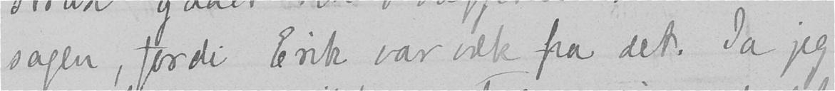sagen, fordi Erik var væk fra det. Ja jeg
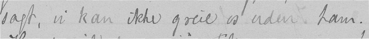sagt, vi kan ikke greie os uden ham.
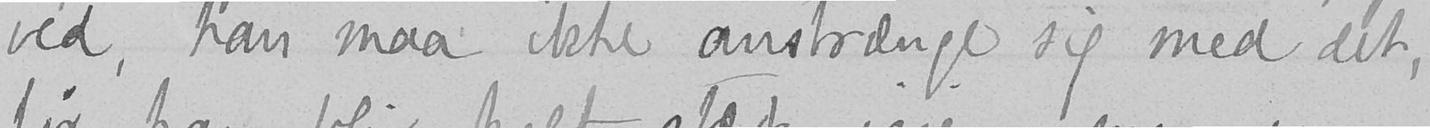ved, han maa ikke anstrænge sig med det,
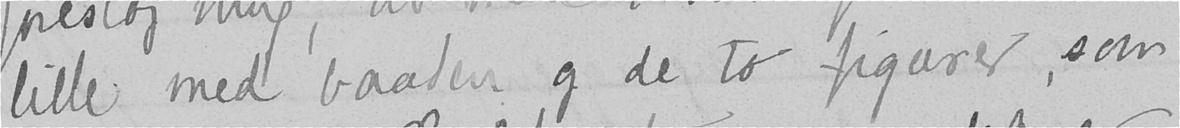lille med baaden og de to figurer, som
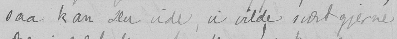saa kan Du vide, vi vilde svært gjerne
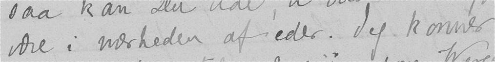være i nærheden af eder. Jeg kommer
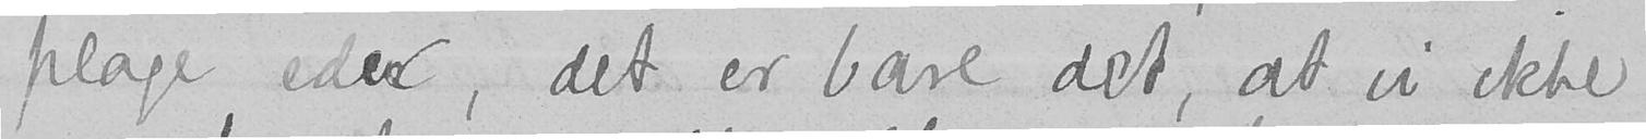plage eder, det er bare det, at vi ikke
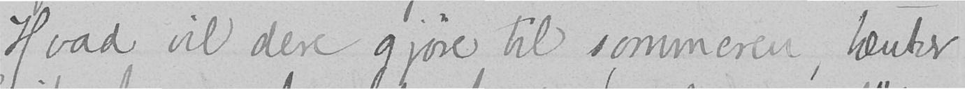Hvad vil dere gjøre til sommeren, tænker
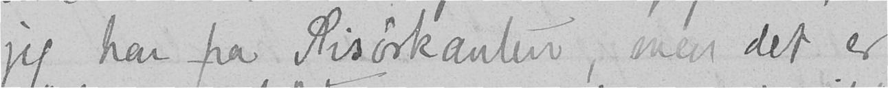jeg har fra Risørkanten, men det er
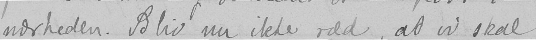nærheden. Bliv nu ikke ræd, at vi skal
